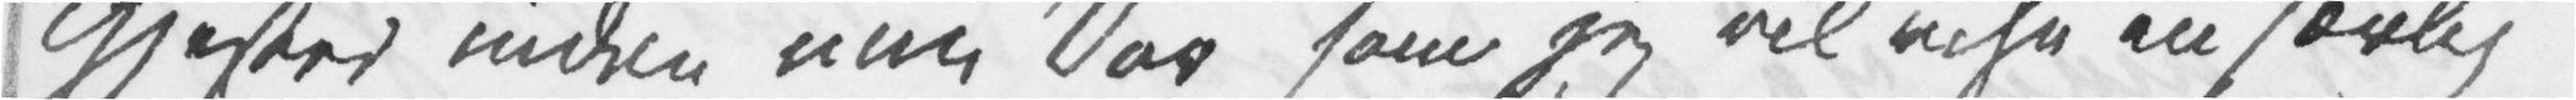Gjester inden min Dør som jeg vil vise en særlig
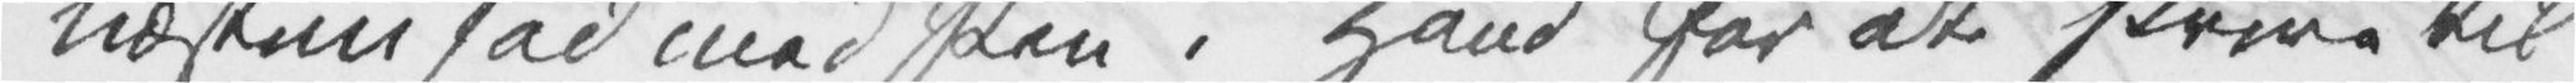næsten sad med Pen i Hånd for at skrive til
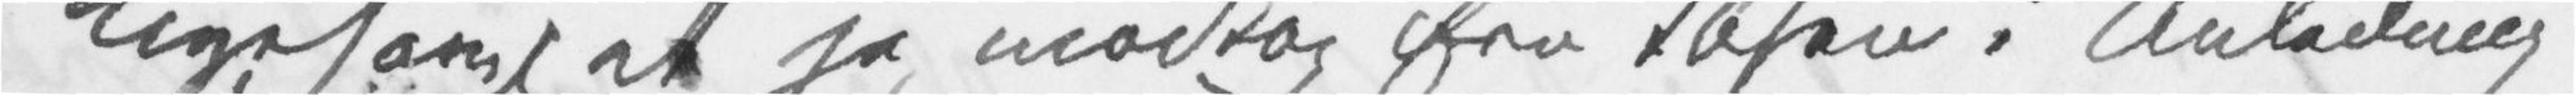ligesom et jeg modtog fra Ibsen i Anledning
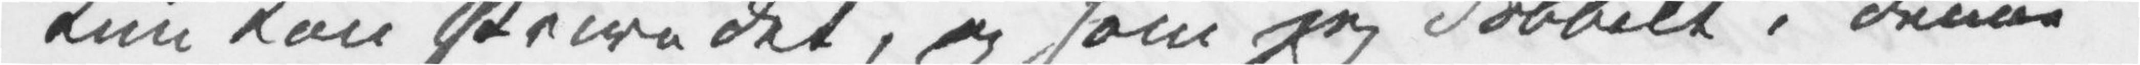kun kan skrive det, og som jeg dobbelt i denne
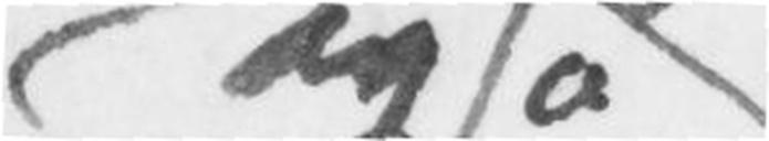også
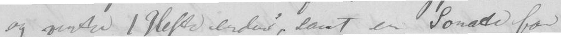og venter 1 Hefte endnu, samt en Sonate for
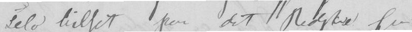selv hilset paa det Bedste fra
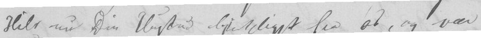Hils nu Din Hustru hjerteligst fra os, og vær
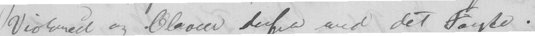Violonell og Claver derfra med det Første.
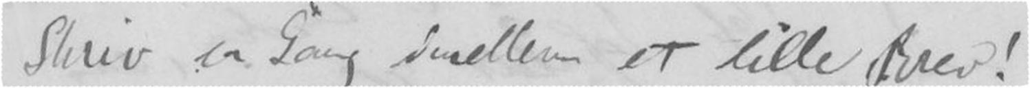Skriv en Gang imellem et lille Brev!
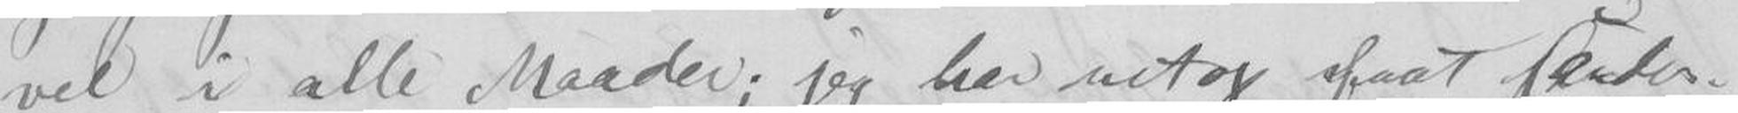vel i alle Maader; jeg har netop faat sende-
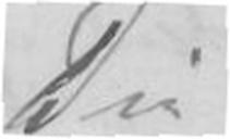Din
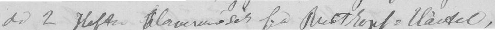de 2 Hefter Clavermusik fra Breitkopf & Härtel,
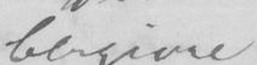hengivne
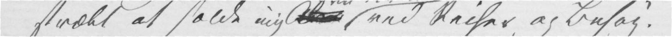stræbt at holde mig A<gap /></del> ved Reiser og Besøg.
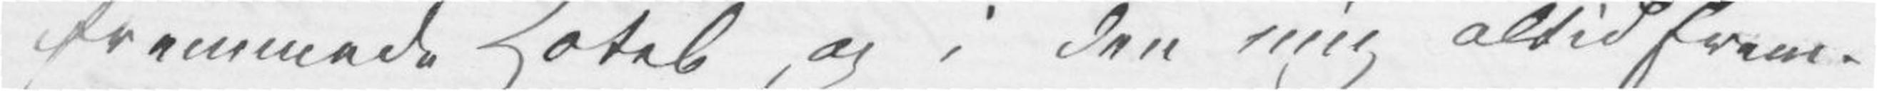fremmede Hotel og i den mig altid frem¬
